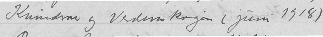Kvinderne og Verdenskrigen (juni 1918)
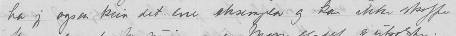har jeg ogsaa kun det ene eksemplar og kan ikke skaffe
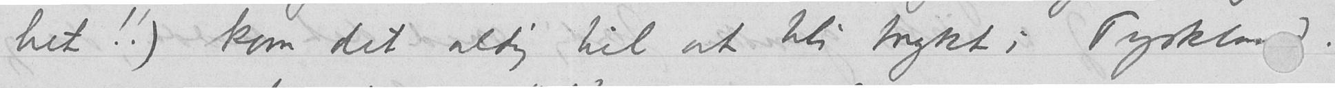het!!) kom det aldrig til at bli trykt i Tyskland.
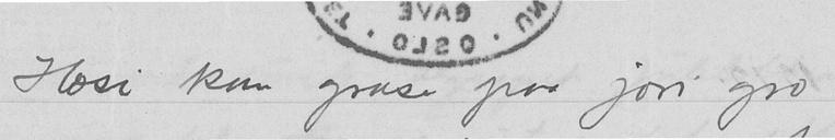Hosi kan grase paa jori gro
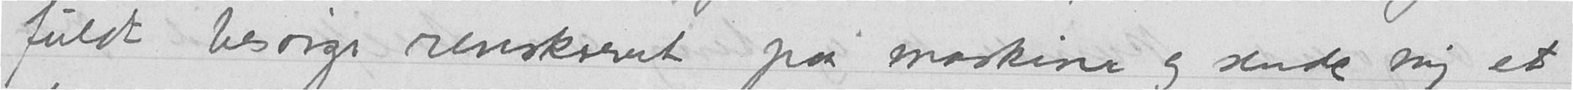fuldt besørge renskrevet paa maskine og sende mig et
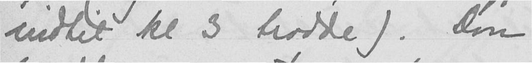indtil kl 3 trodde). Don
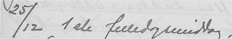25/12 1ste Juledagsmiddag,
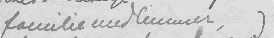familiemedlemmer, og
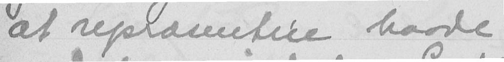at repræsentere baade
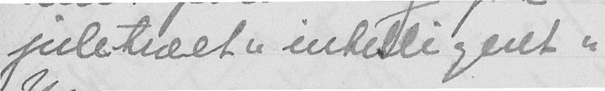juletræet "intelligent"
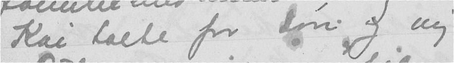Kai talte for Don og mig
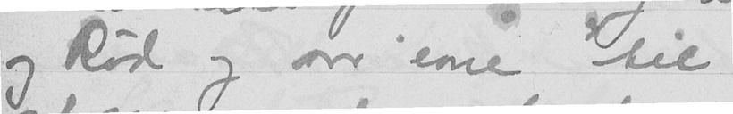og Rød og vor evne til
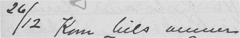26/12 Kom Nils venner
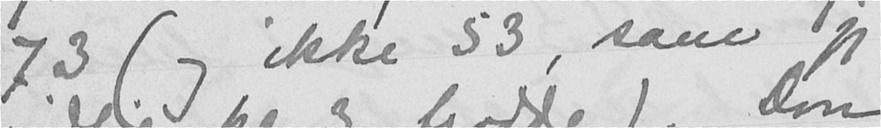73 (og ikke 53, som jeg
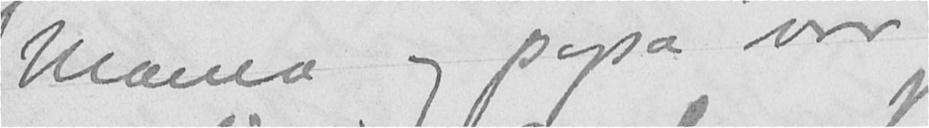Mama og papa var
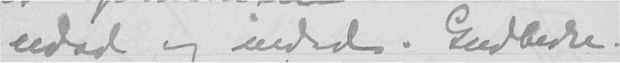udad og indad. Gudbedre.
